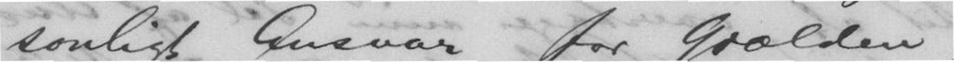sonligt Ansvar for Gjælden,
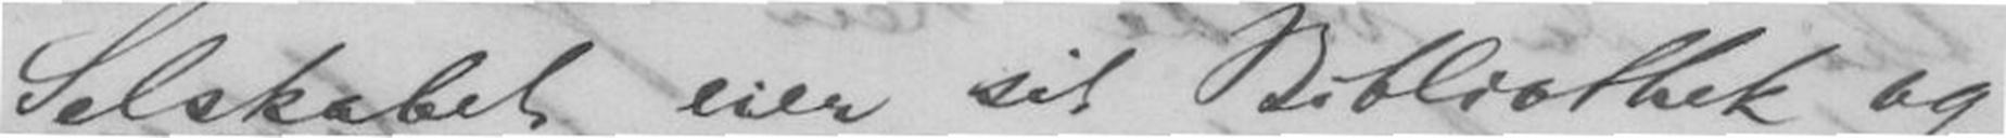Selskabet eier sit Bibliothek og
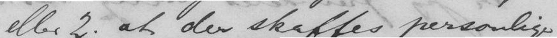eller 2. at der skaffes personlige
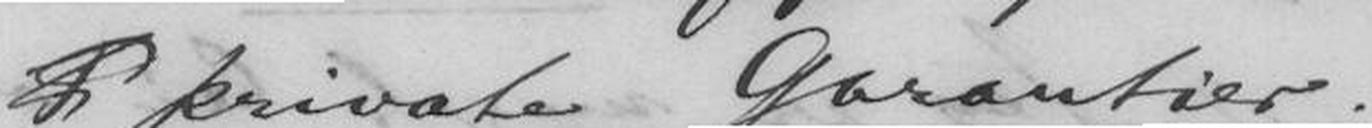P private Garantier.
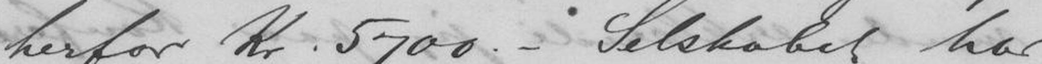herfor Kr. 5700. - Selskabet har
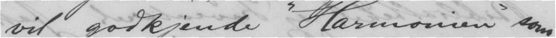vil godkjende "Harmonien" som
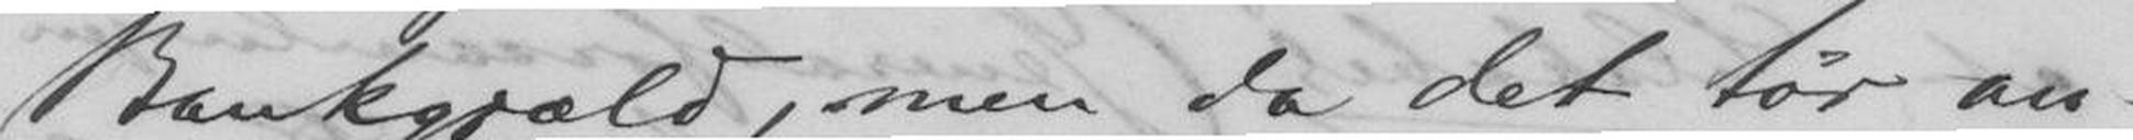Bankgjæld, men da det tør an-
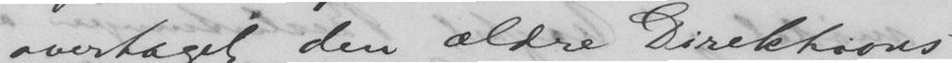overtaget den ældre Direktions
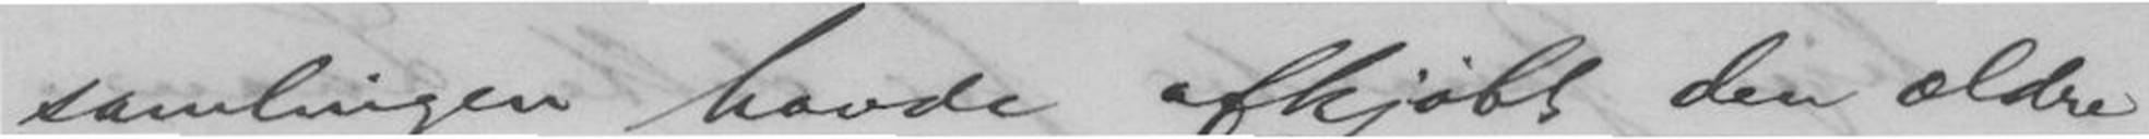samlingen havde afkjøbt den ældre
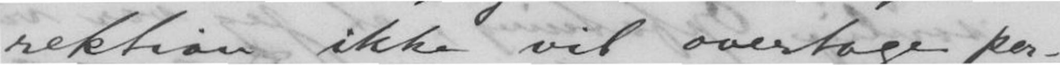rektion ikke vil overtage per-
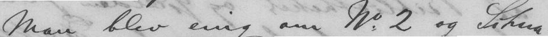Man blev enig om N.. 2 og Situa-
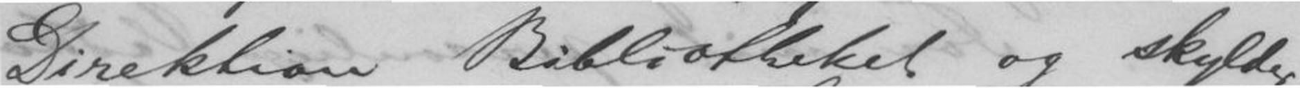Direktion Bibliotheket og skylder
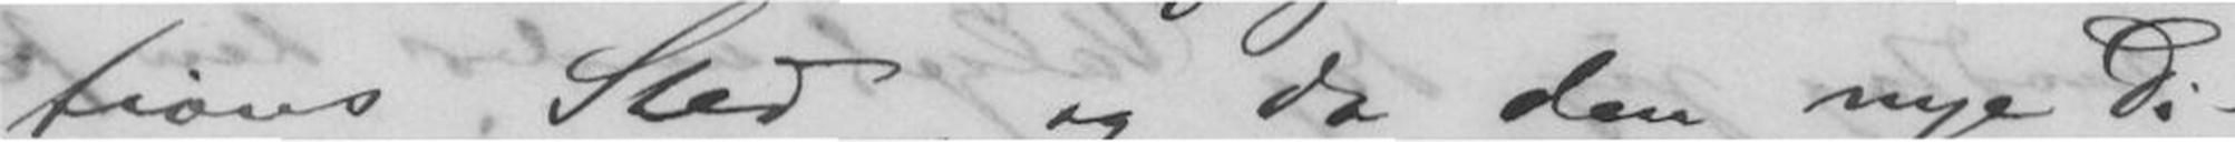tions Sted, og da den nye Di-
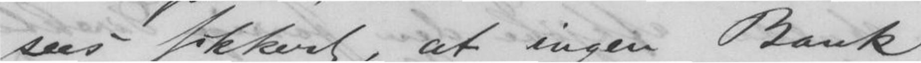sees sikkert, at ingen Bank
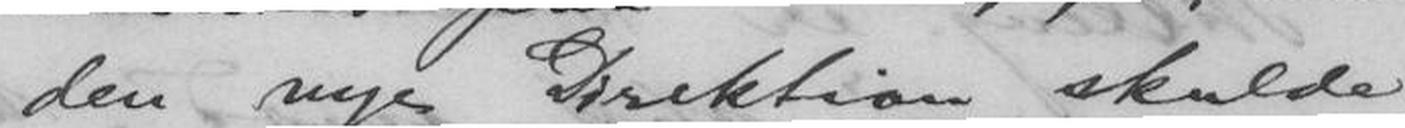den nye Direktion skulde
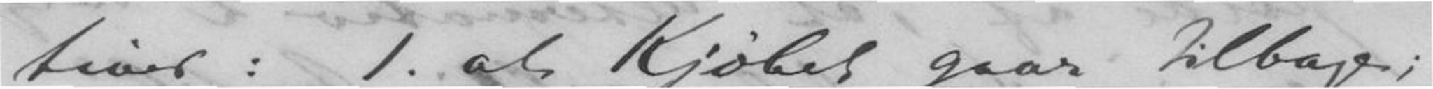tiver: 1. at Kjøbet gaar tilbage;
